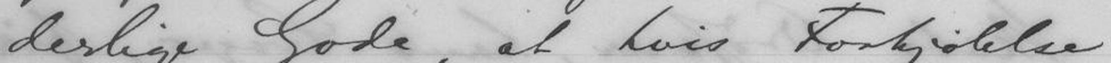derlige Gode, at hvis Forkjølelse
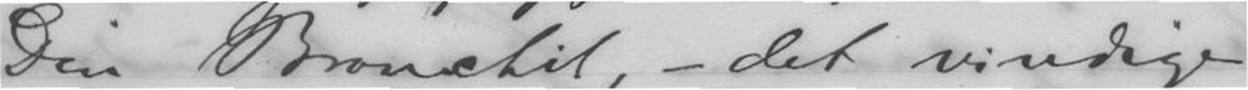Din Bronchit, - det vindige,
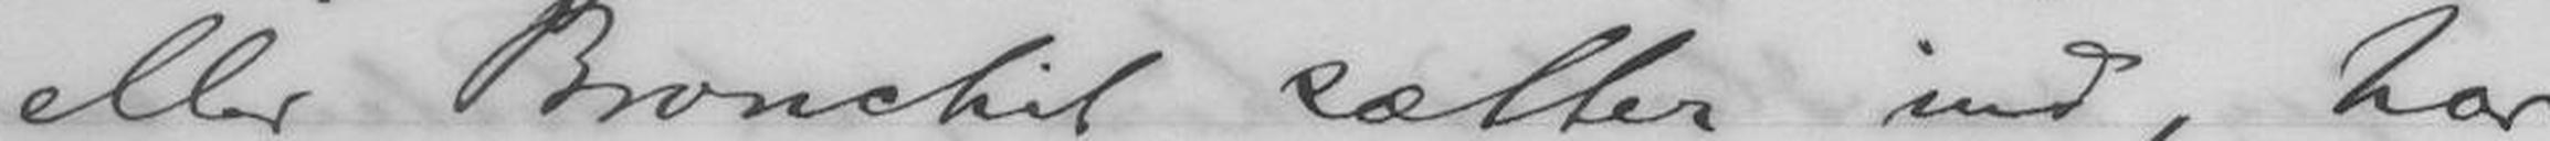eller Bronchit sætter ind, har
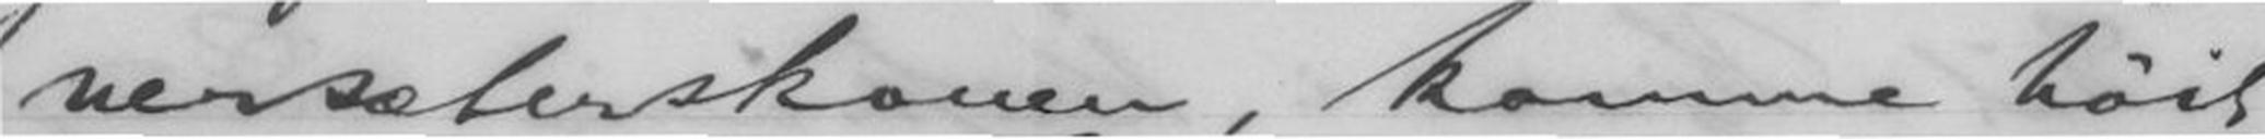nersæterskouen, komme høit
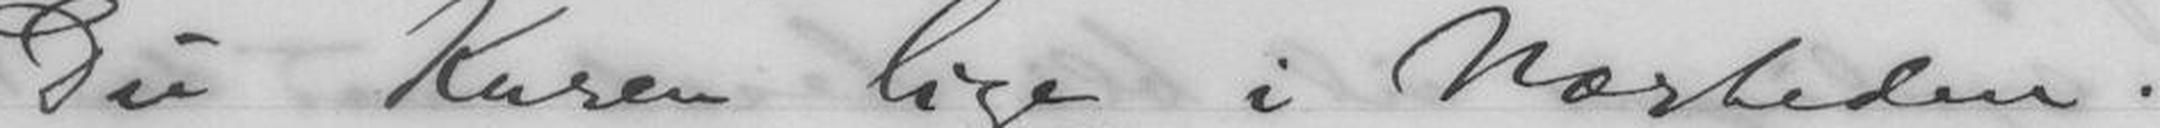Du Kuren lige i Nærheden.
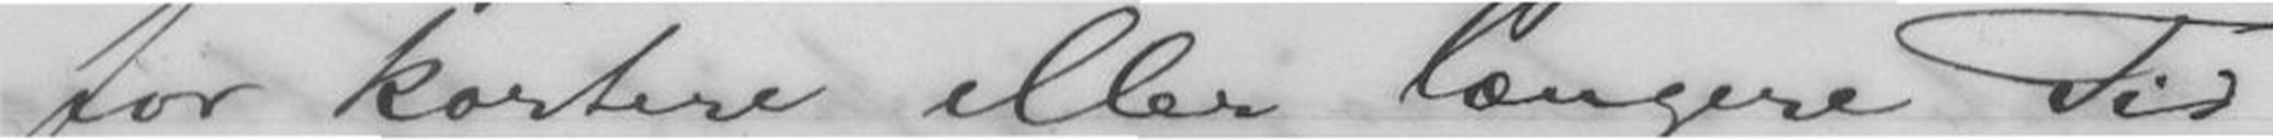for kortere eller længre Tid;
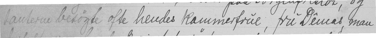tanterne besøgde ofte hendes kammerfrue, fru Dêmas, man
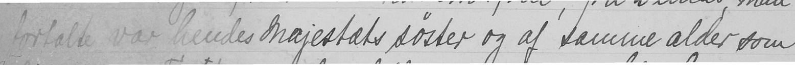fortalte var hendes majestæts søster og af samme alder som
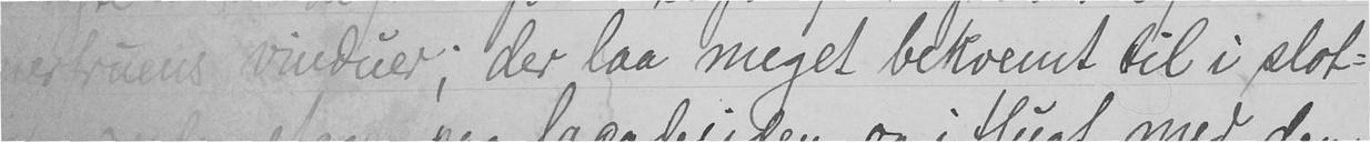merfruens vinduer; der laa meget bekvemt til i slot-
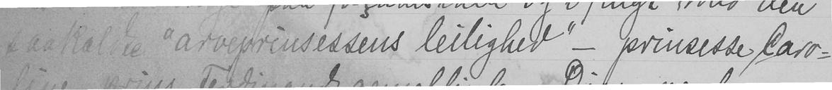saakalde "arveprinsessens leilighed" - prinsesse Caro-
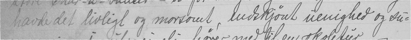havde det livligt og morsomt, endskjønt uenighed og su-
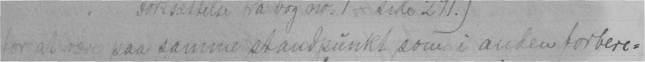For at være paa samme standpunkt som i anden forbere-
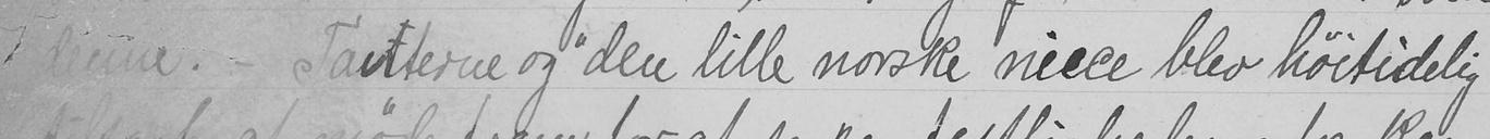denne. - Tanterne og "den lille norske niece blev høitidelig
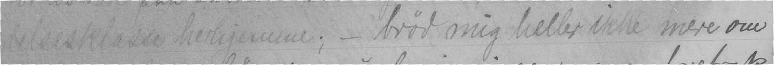delsesklasse her hjemme; -  brød mig heller ikke mere om
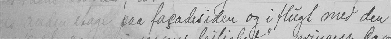tets anden etage paa façadesiden og i flugt med den
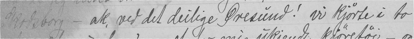Sarpsborg - ak, ved det derlige Øresund! Vi kjørte i to
